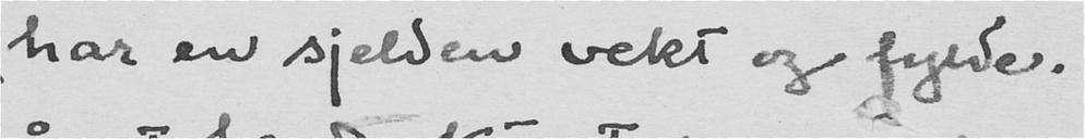har en sjelden vekt og fylde.
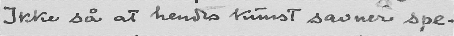Ikke så at hendes kunst savner spe-
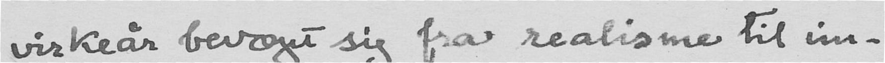virkeår bevæget sig fra realisme til im-
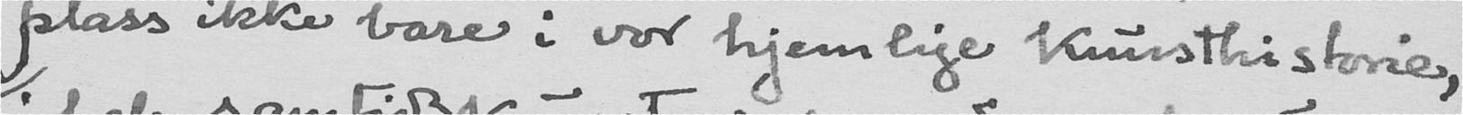plass ikke bare i vor hjemlige kunsthistorie,
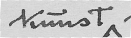kunst
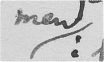men
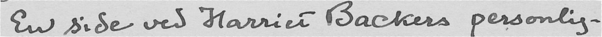En side ved Harriet Backers personlig-
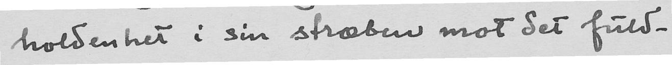holdenhet i sin stræben mot det fuld-
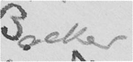Backer
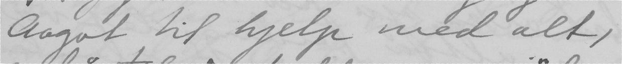Aagot til hjelp med alt,
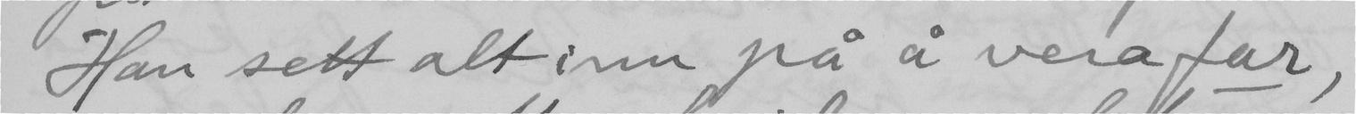Han sett alt inn på å vera far,
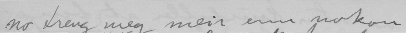no treng meg meir enn nokon
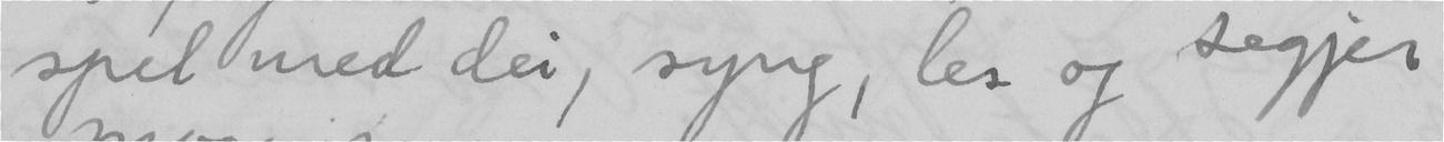spel med dei, syng, les og segjer
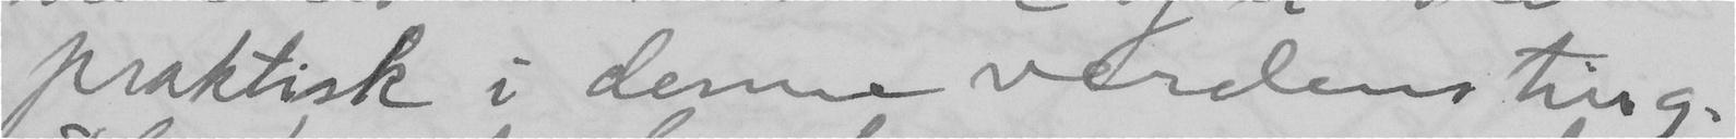praktisk i denne verdens ting.
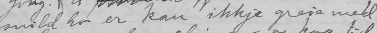snild ho er kan ikkje greie med
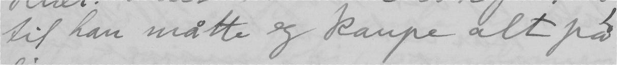til han måtte og kaupe alt på
